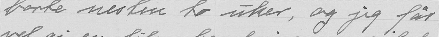borte nesten to uker, og jeg får
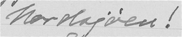Nordsjøen!
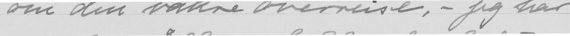om din vakre overreise, - jeg har
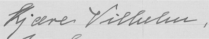Kjære Vilhelm,
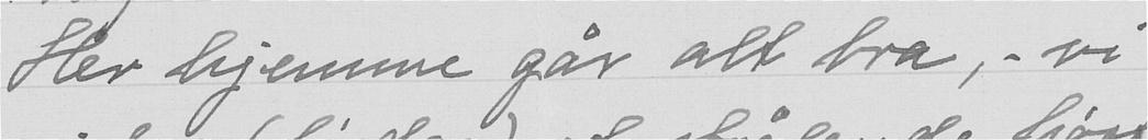Her hjemme går alt bra, - vi
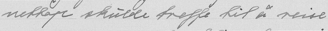nettop skulde treffe til å reise
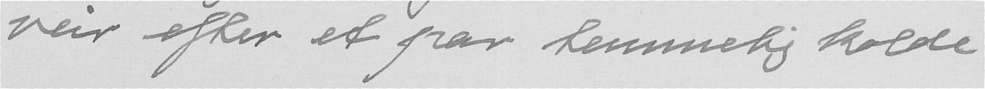veir efter et par temmelig kolde
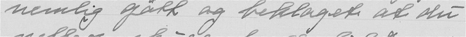nemlig gått og beklaget at du
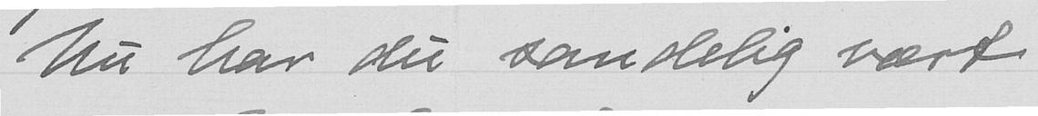Nu har du sandelig vært
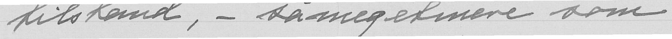tilstand, - så megetmere som
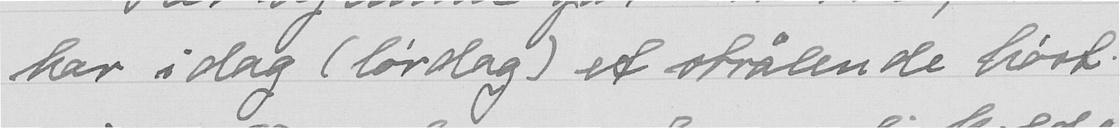har i dag (lørdag) et strålende høst-
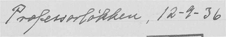Professorløkken, 12-9-36
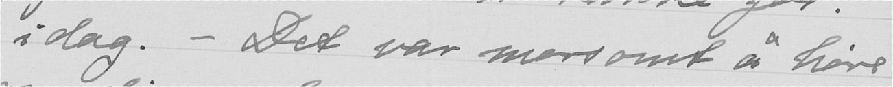i dag. - Det var morsomt å høre
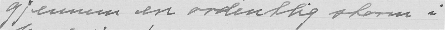gjennem en ordentlig storm i
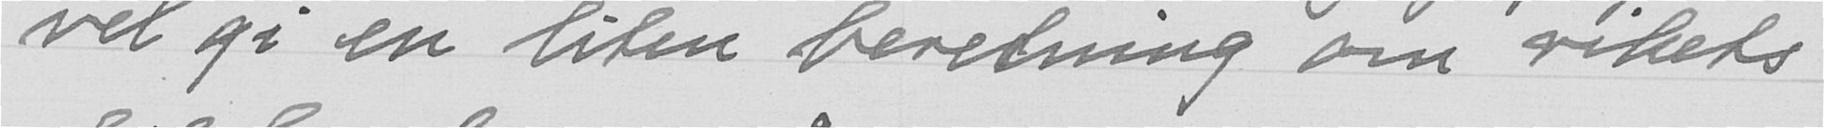vel gi en liten beretning om rikets
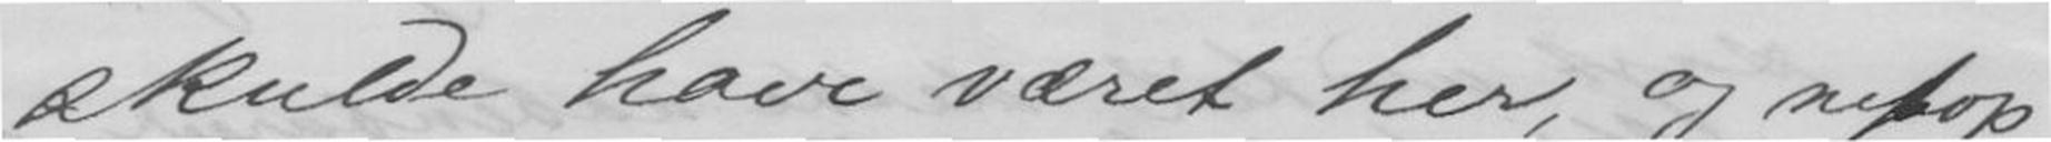skulde have været her, og netop
